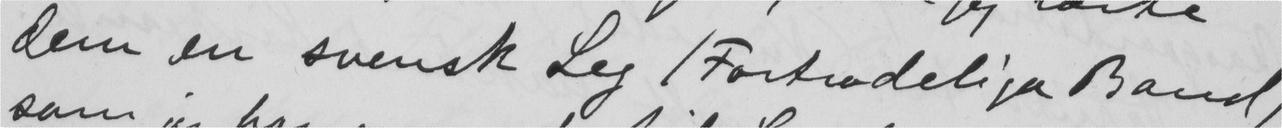dem en svensk Leg (Fortrodeliga Band)
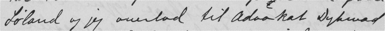Løland og jeg overlod til Advokat Dybwad
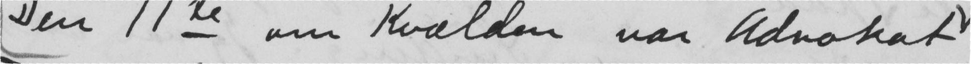Den 11te om Kvælden var Advokat
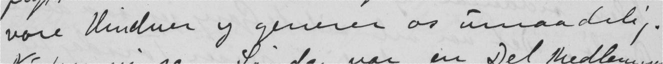vore Vinduer og generer os umaadelig.
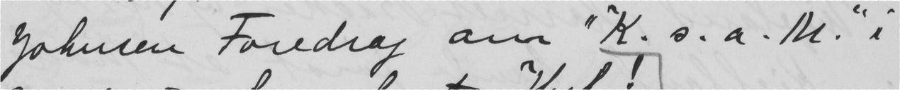Johnsen Foredrag om "K. s. a. M." i
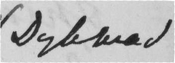Dybwad
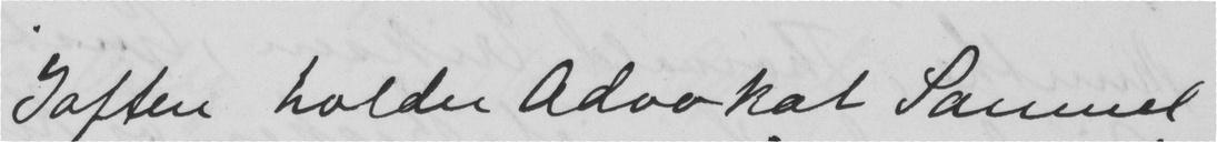Iaften holder Advokat Samuel
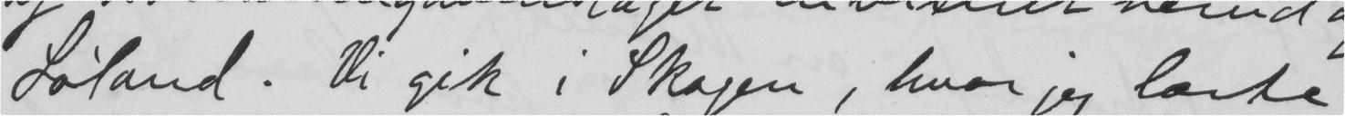Løland. Vi gik i Skogen, hvor jeg lærte
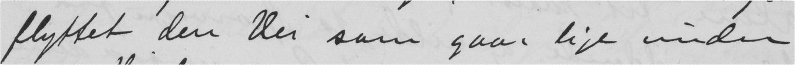flyttet den Vei som gaar lige under
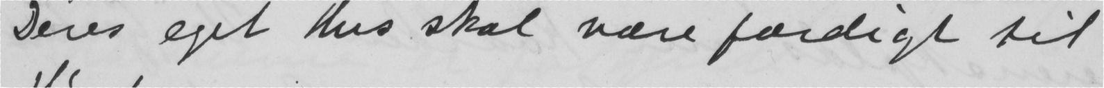Deres eget Hus skal være færdigt til
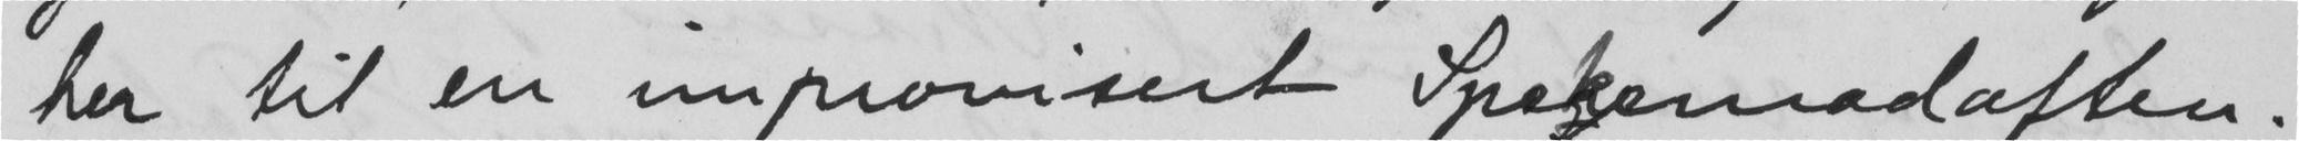her til en improvisert Spekemadaften.
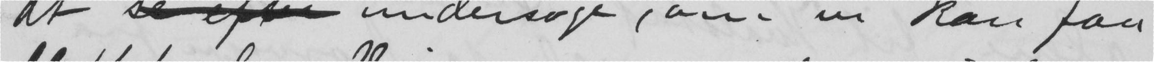at  undersøge, om vi kan faa
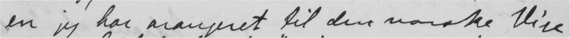en jeg har arangeret til den vorske Vise
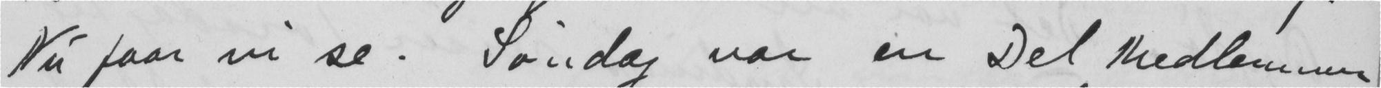Nu faar vi se. Søndag var en Del Medlemmer
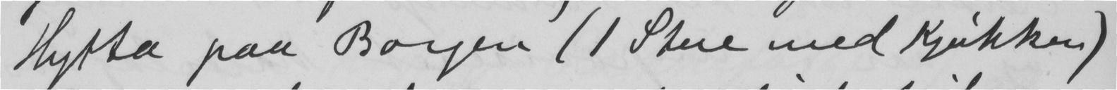Hytta paa Borgen (1 Stue med Kjøkken)
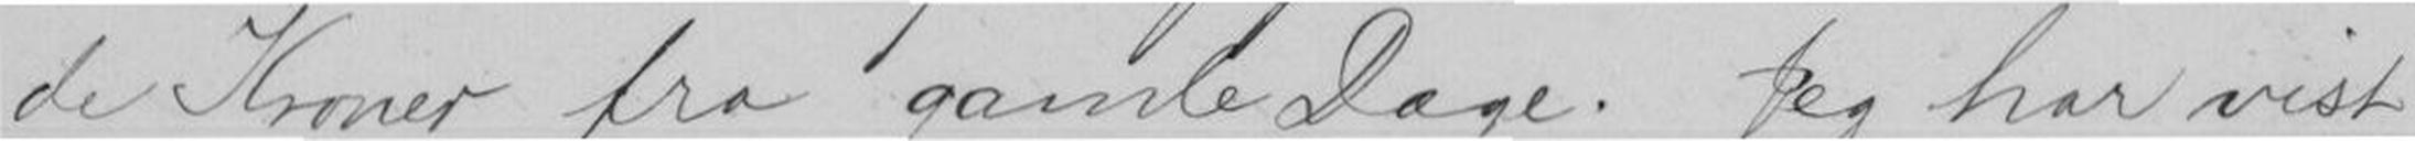de Kroner fra gamle Dage. Jeg har vist
